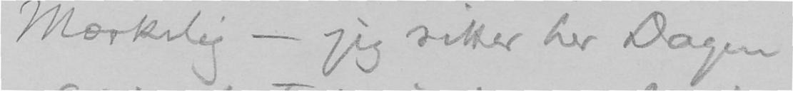Mærkelig - jeg sitter her Dagen
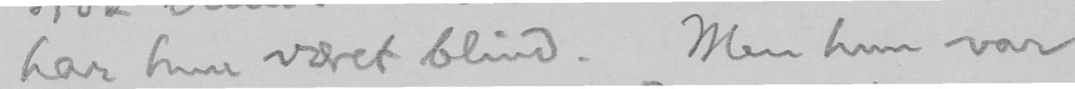har hun været blind. Men hun var
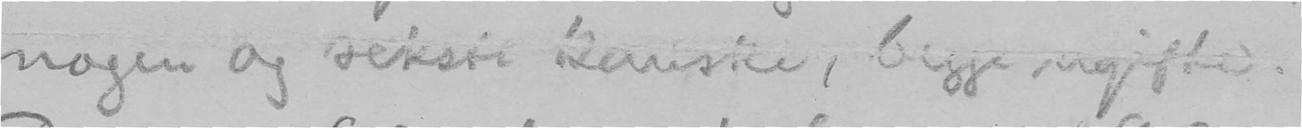nogen og seksti kanske, begge ugifte.
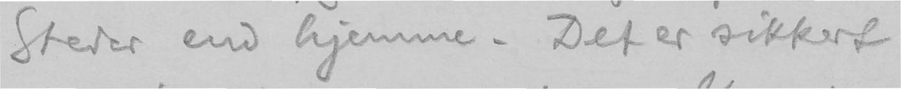Steder end hjemme. Det er sikkert
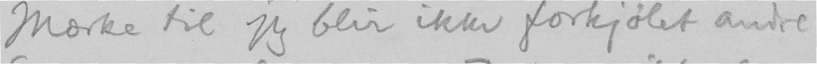Mærke til jeg blir ikke forkjølet andre
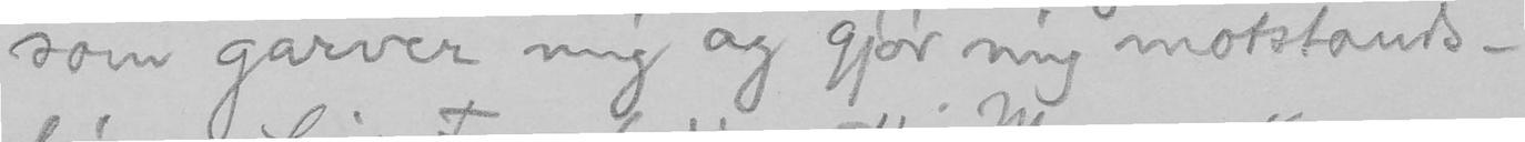som garver mig og gjør mig motstands-
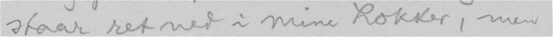staar ret ned i mine Lokker, men
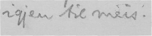igjen til meis.
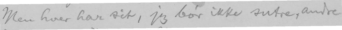Men hver har sit, jeg bør ikke sutre, andre
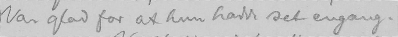Var glad for at hun hadde set engang.
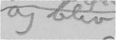og blev
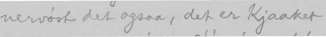nervøst det ogsaa, det er Kjaaket
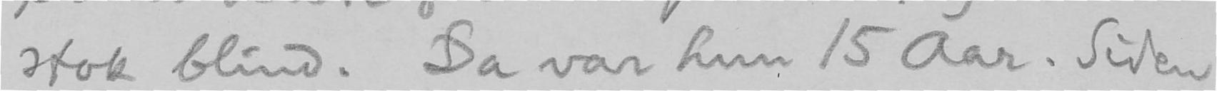stok blind. Da var hun 15 Aar. Siden
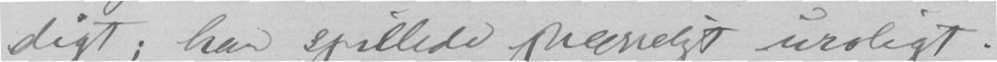digt; han spillede skrekkeligt uroligt.
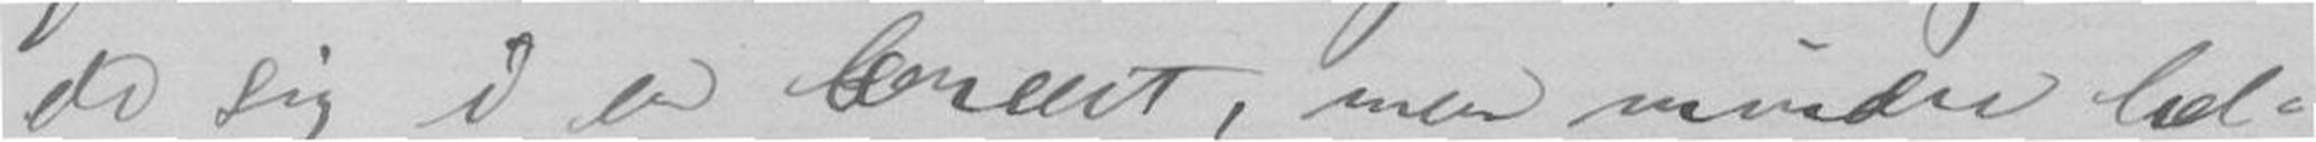de sig i en Concert, men mindre hel-
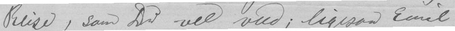Reise, som Du vel veed; ligesom Emil
